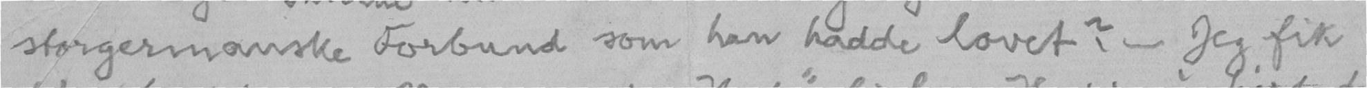storgermanske Forbund som han hadde lovet? - Jeg fik
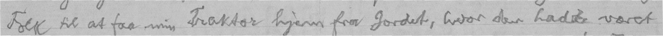Folk til at faa min Traktor hjem fra Jordet, hvor den hadde været
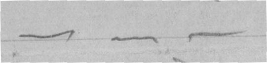- - -
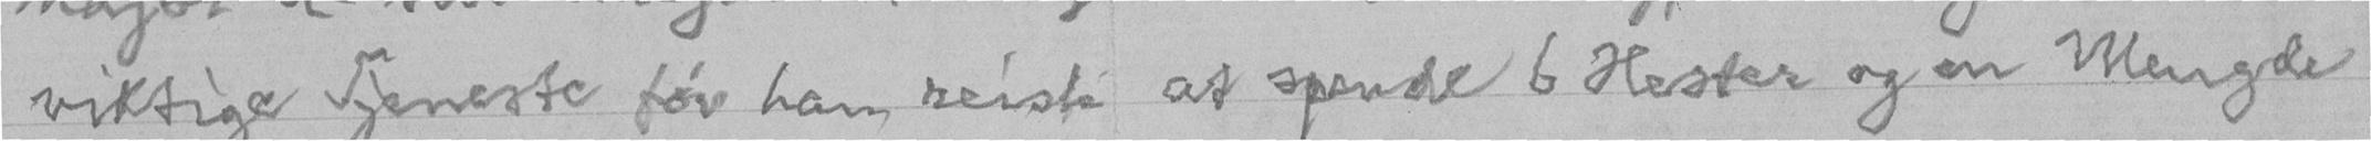viktige Tjeneste før han reiste at spende 6 Hester og en Mengde
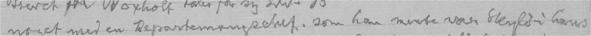noget med en Departemangschef, som han mente var skyld i hans
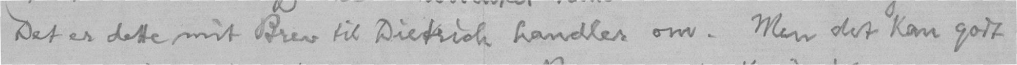Det er dette mit Brev til Dietrich handler om. Men det kan godt
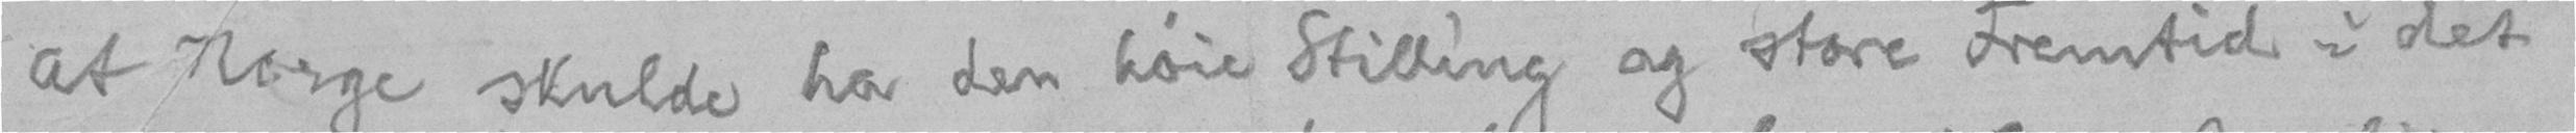at Norge skulde ha den høie Stilling og store Fremtid i det
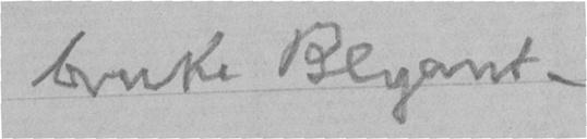bruke Blyant.
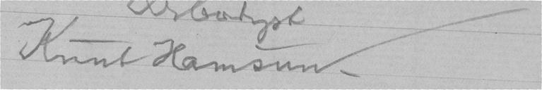Knut Hamsun.
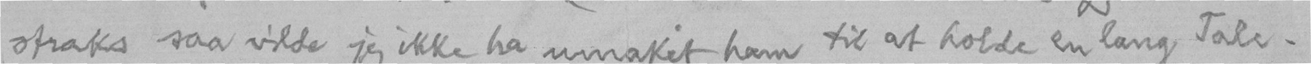straks saa vilde jeg ikke ha umaket ham til at holde en lang Tale.
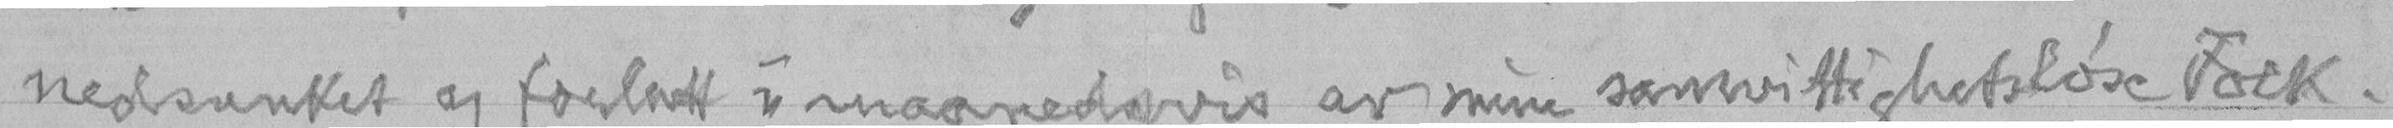nedsunket og forlatt i maanedsvis av mine samvittighetsløse Folk.
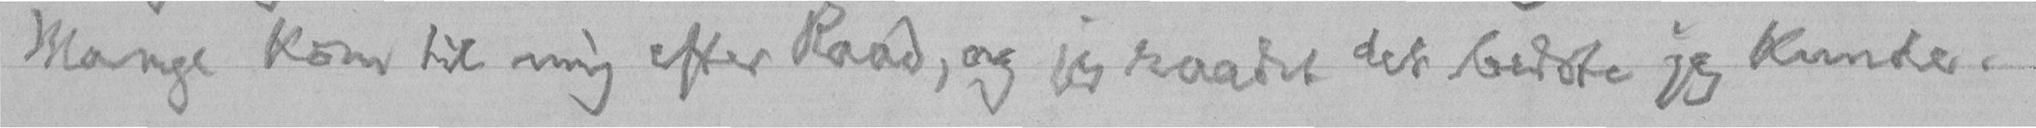Mange kom til mig efter Raad, og jeg raadet det bedste jeg kunde.
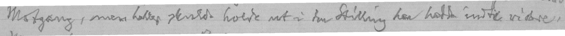Motgang, men heller skulde holde ut i den Stilling han hadde indtil videre.
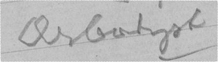Ærbødigst
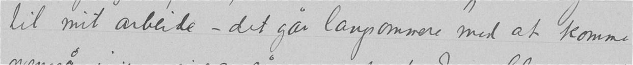til mit arbeide – det går langsommere med at komme
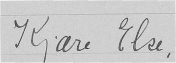Kjære Else,
